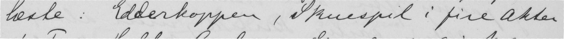læste: Edderkoppen, Skuespil i fire Akter
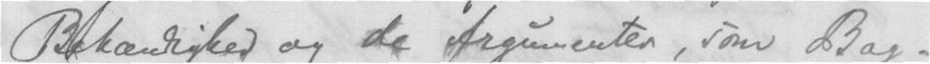Bekændlighed og de Argumenter, som Bag-
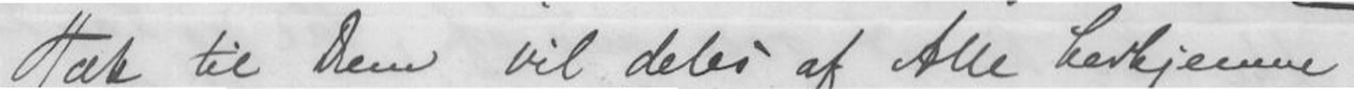Tak til Dem vil deles af Alle herhjemme,
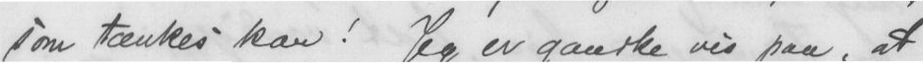som tænkes kan ! Jeg er ganske vis paa, at
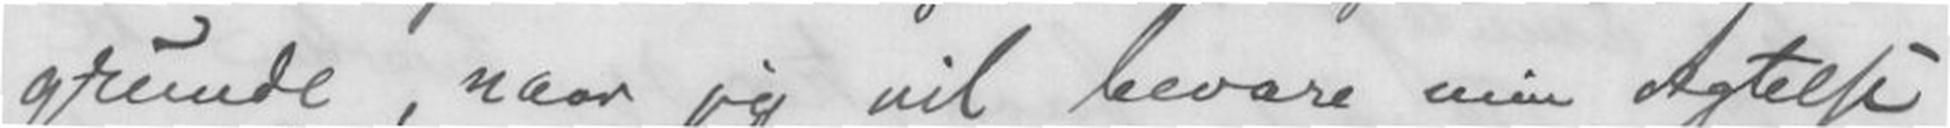grunde, naar jeg vil bevare min Agtelse
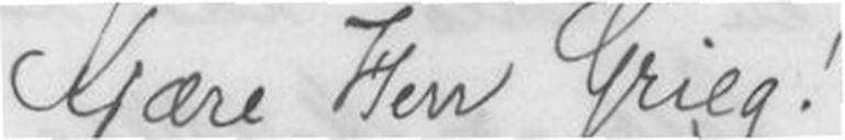Kjære Herr Grieg !
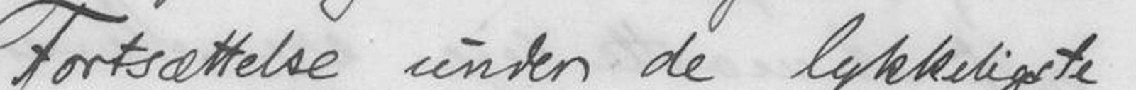Fortsættelse inden de lykkeligste
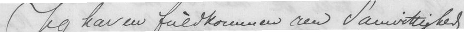Jeg har en fuldkommen ren Samvittighed
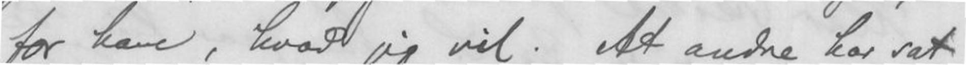for ham, hvad jeg vil. At andre har sat
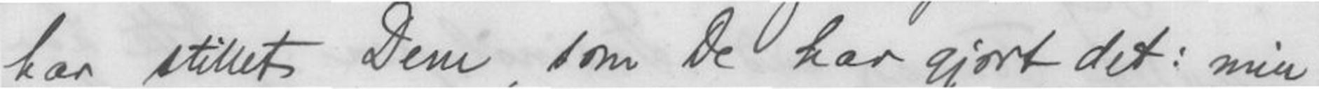har stillet dem, som De har gjort det: min
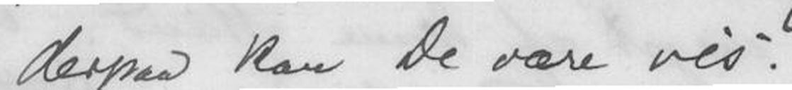derpaa kan De være vis !
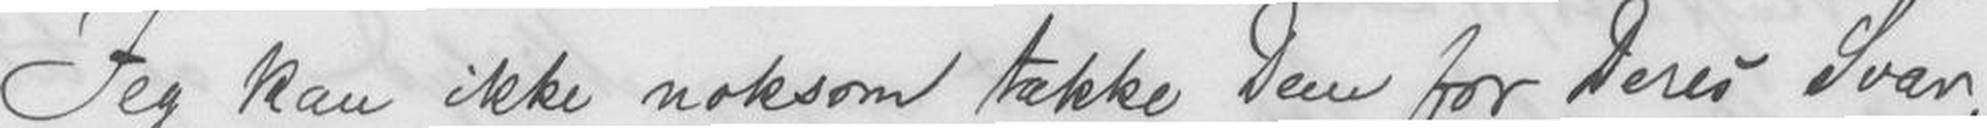Jeg kan ikke noksom takke Dem for Deres Svar,
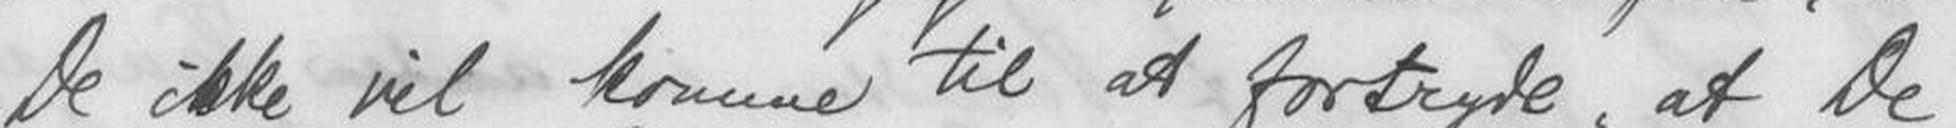De ikke vil komme til at fortryde, at De
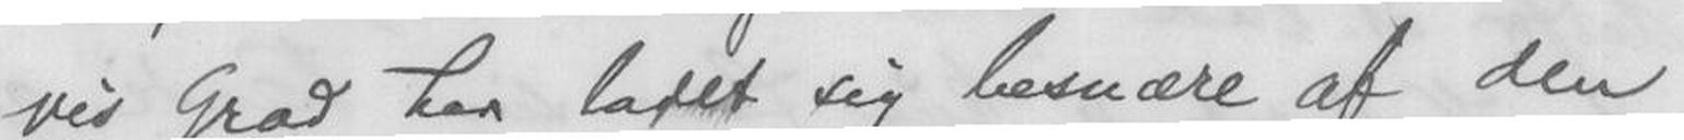vis Grad har lafet sig besnære af den
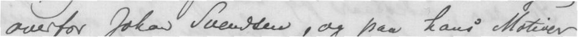overfor Johan Svendsen, og paa hans Motiver
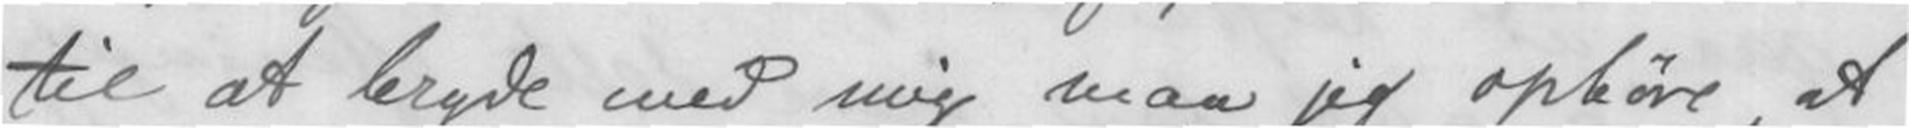til at med mig man jeg ophøre at
